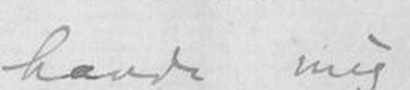havde mig
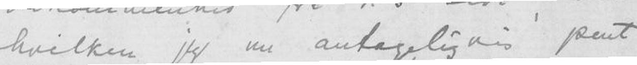hvilken jeg nu antageligvis pent
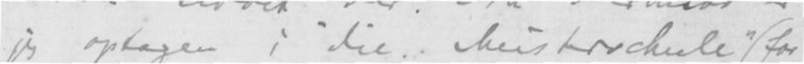jeg optagen i "die Meisterchule" (for
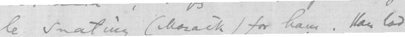le småting (Mozaik) for ham. Han lod
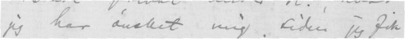jeg har ønsket mig siden jeg fik
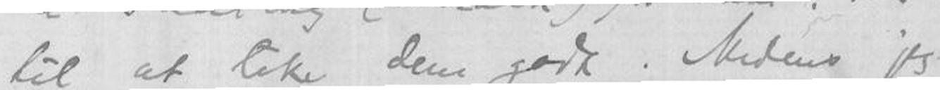til at like dem godt. Medens jeg
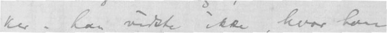ker - han vidste ikke hvor han
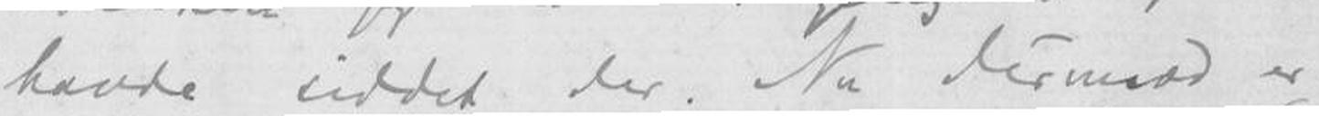havde siddet der. Nu derimod er
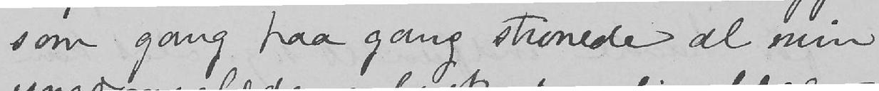som gang paa gang stivnede al min
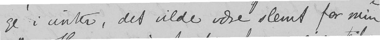ge i vinter, det vilde være slemt for min
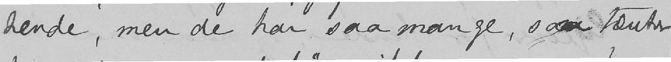hende, men de har saa mange, som tænker
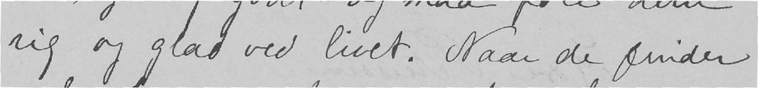rig og glad ved livet. Naar de finder
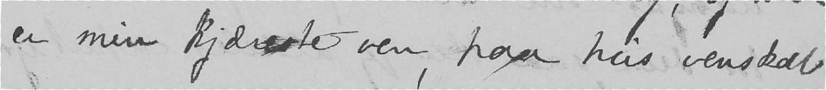er min kjæreste ven, paa hvis venskab
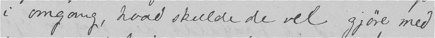i omgang, hvad skulde de vel gjøre med
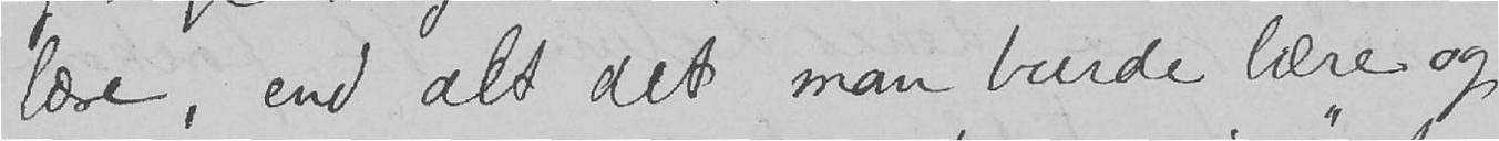lære, end alt det man burde lære og
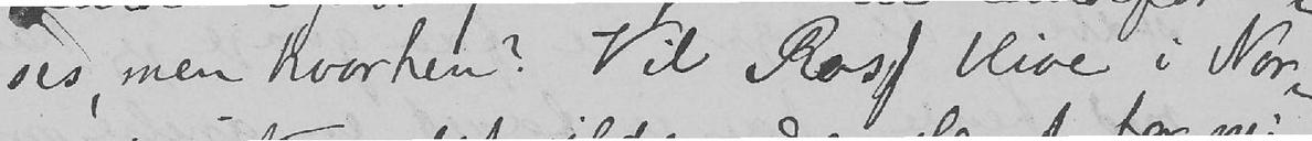ses, men hvorhen? Vil Ross blive i Nor-
